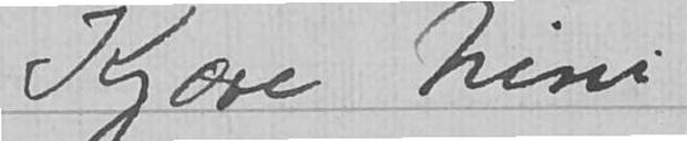Kjære Nini,
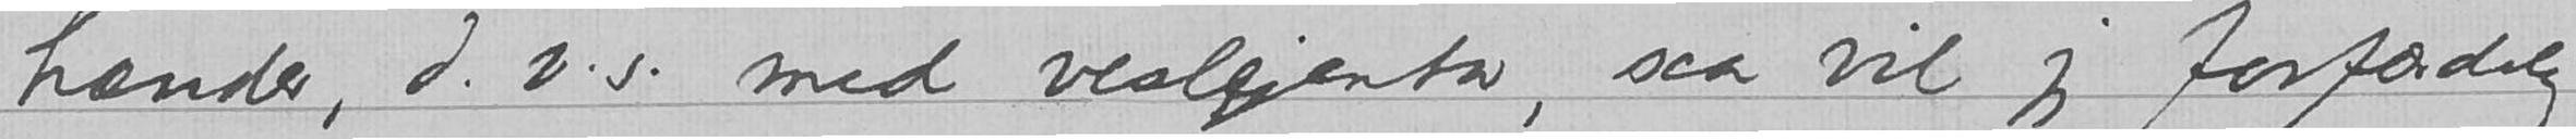hender, d.v.s. med veslejenta, saa vil jeg forfærdelig
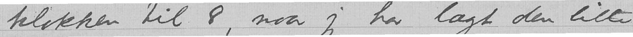klokken til 8, naar jeg har lagt den lille
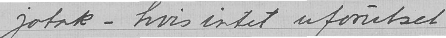jotak – hvis intet uforutset
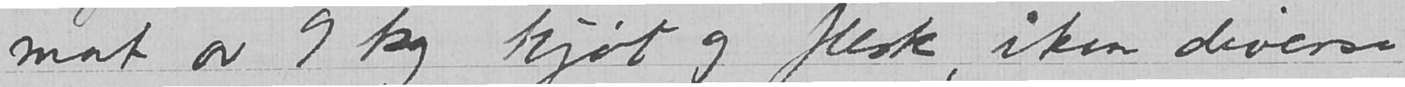mat av 9 kg kjøt og flesk, item diverse
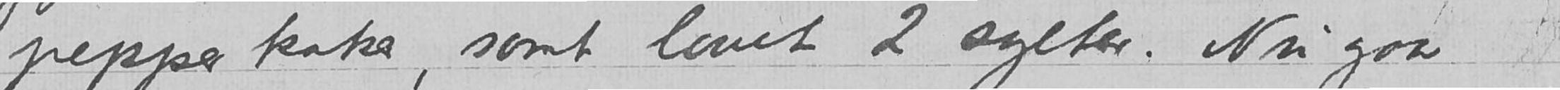pepperkaker, samt lavet 2 sylter. Nu gaar
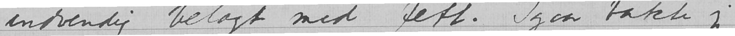indvendig belagt med fett. Igaar bakte jeg
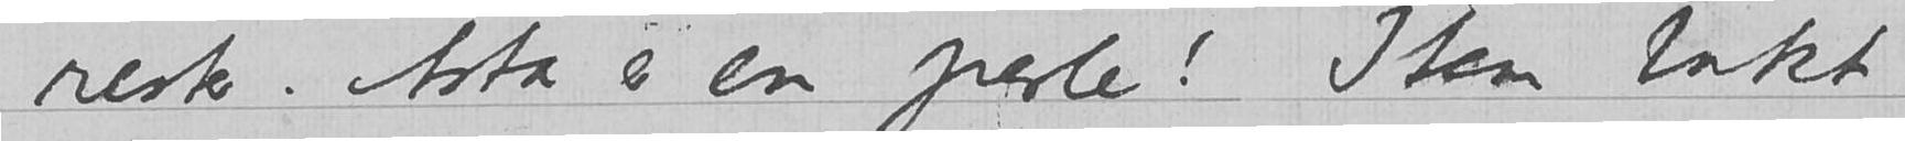rask. Asta er en perle! Item bakt
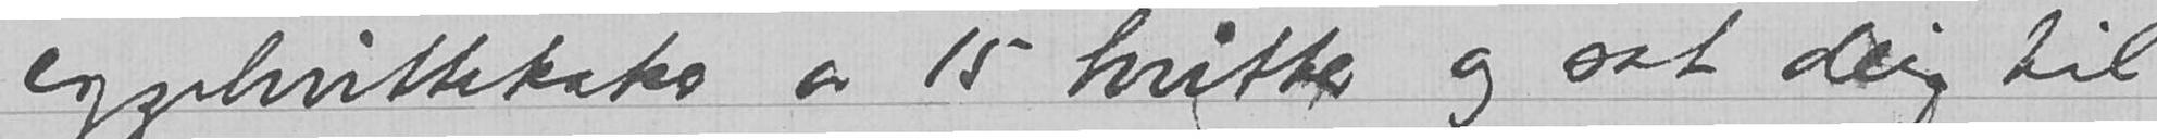eggehvittekaker av 15 hvitter og sat deig til
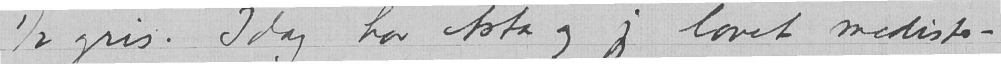½ gris. Idag har Asta og jeg lavet medister
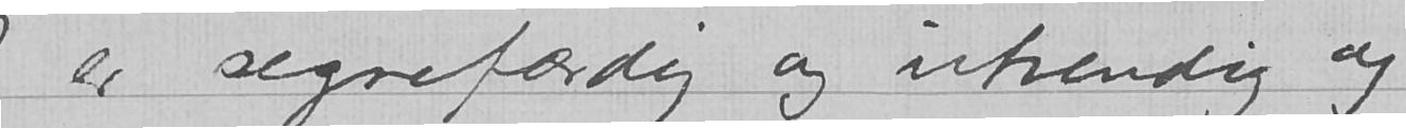Jeg er segnefærdig og utvendig og
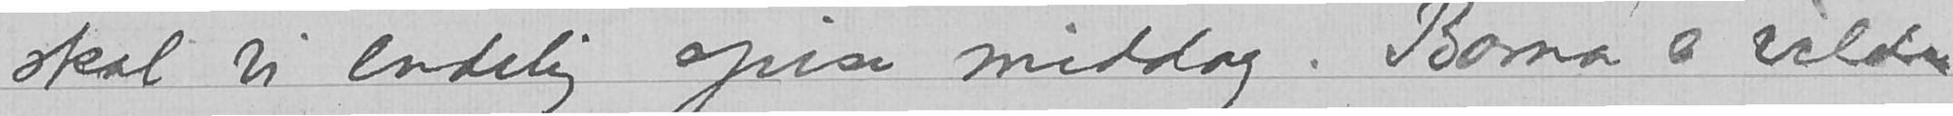skal vi endelig spise middag. Barna er vilde
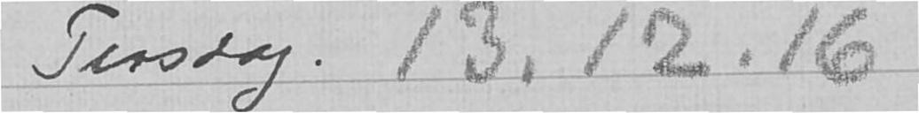Tirsdag 13.12.16
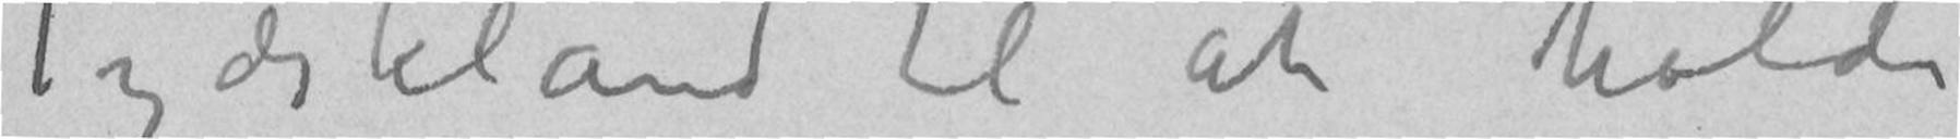Tydskland til at holde
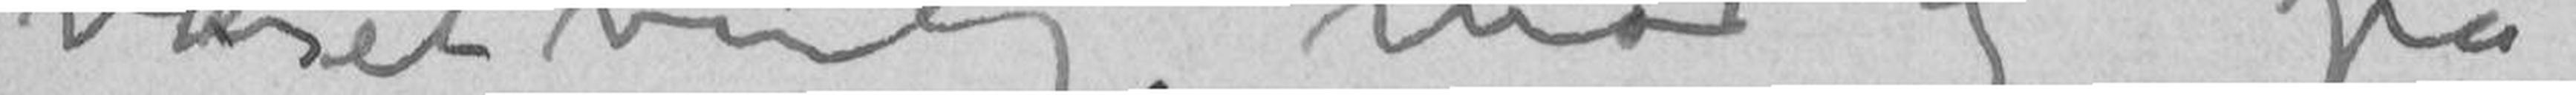været venlig mod og på
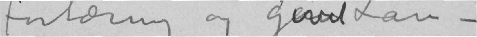Fortæring og givet Lån –
